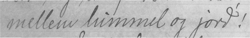mellem himmel og jord!
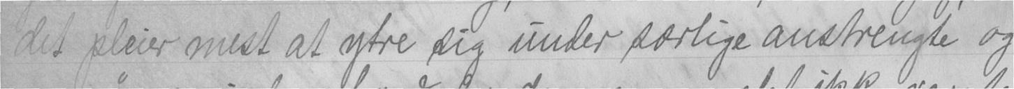det pleier mest at ytre sig under særlige anstrengte og
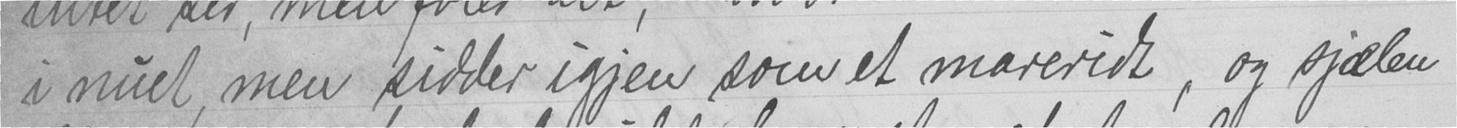i nuet, men sidder igjen som et mareridt, og sjælen
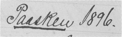Paasken 1896.
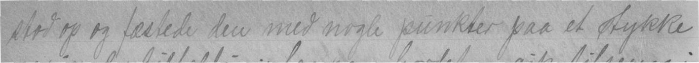stod op og fæstede den med nogle punkter paa et stykke
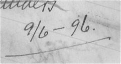9/6-96.
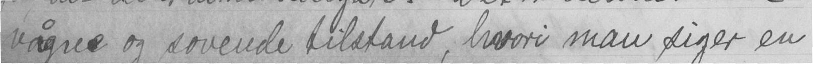vågne og sovende tilstand, hvori man siger en
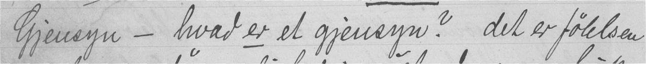Gjensyn - hvad er et gjensyn? det er følelsen
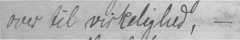over til virkelighed, -
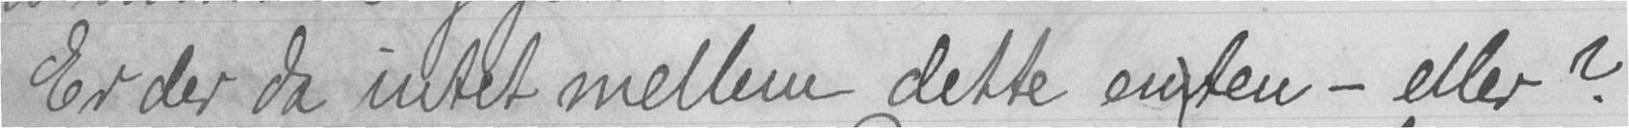Er der da intet mellem dette enten - eller?
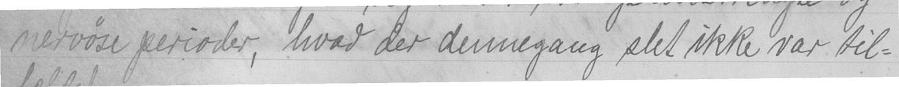nervøse perioder, hvad der dennegang slet ikke var til-
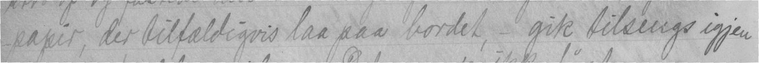papir, der tilfældigvis laa paa bordet, - gik tilsengs igjen
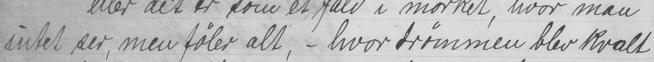intet ser, men føler alt, - hvor drømmen blev kvalt
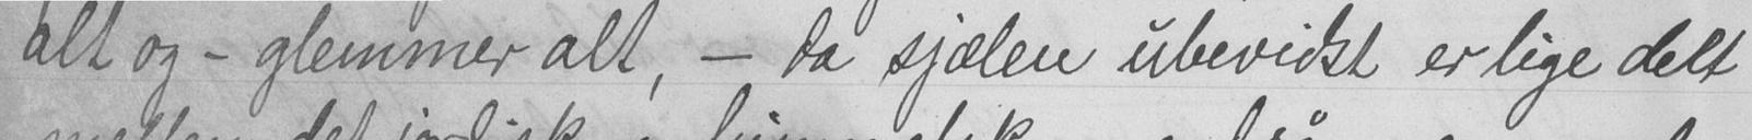alt og - glemmer alt, - da sjælen ubevidst er lige delt
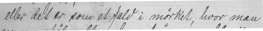eller det er som et fald i mørket, hvor man
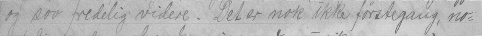og sov fredelig videre. Det er nok ikke førstegang, no-
